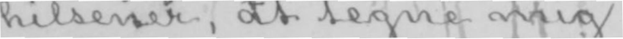hilsener, dat tegne mig
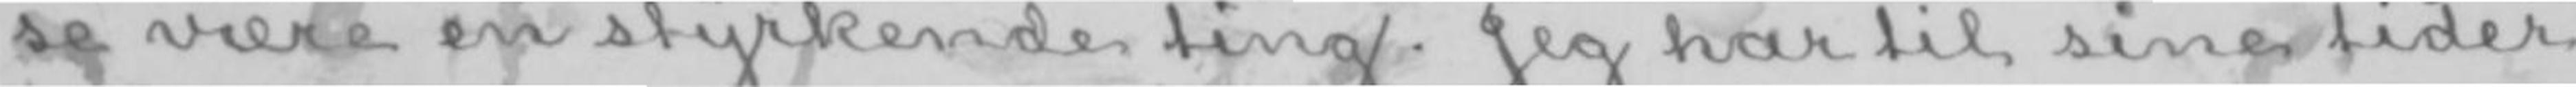se være en styrkende ting. Jeg har til sine tider
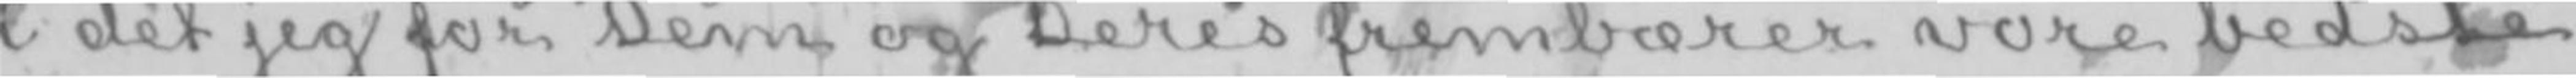i det jeg for Dem og Deres frembærer vore bedste
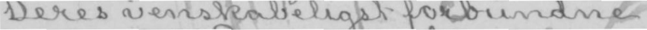Deres venskabeligst forbundne
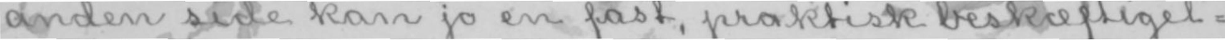anden side kan jo en fast, praktisk beskæftigel-
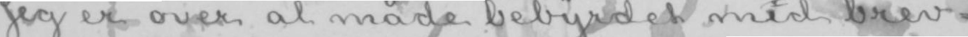Jeg er over al måde bebyrdet med brev-
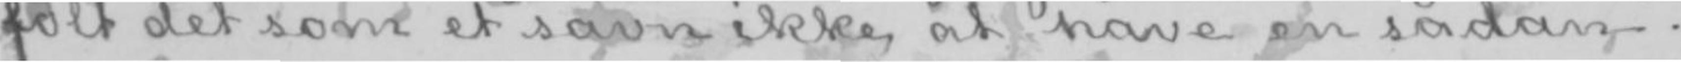følt det som et savn ikke at have en sådan.
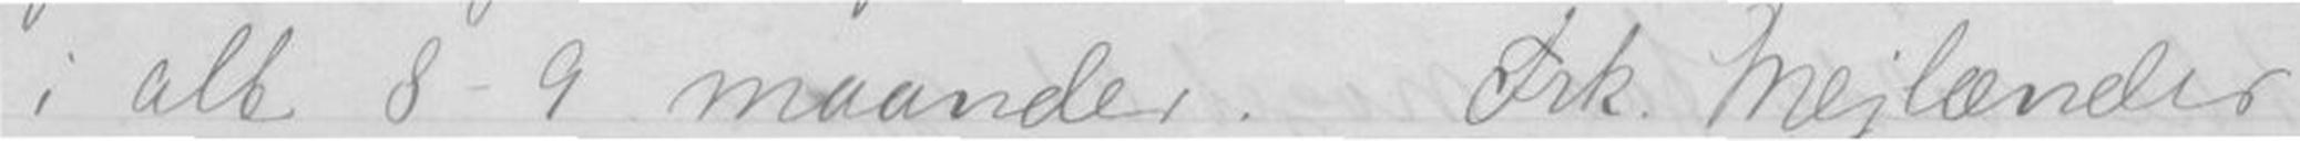i alt 8-9 maander. Frk Mejlænder
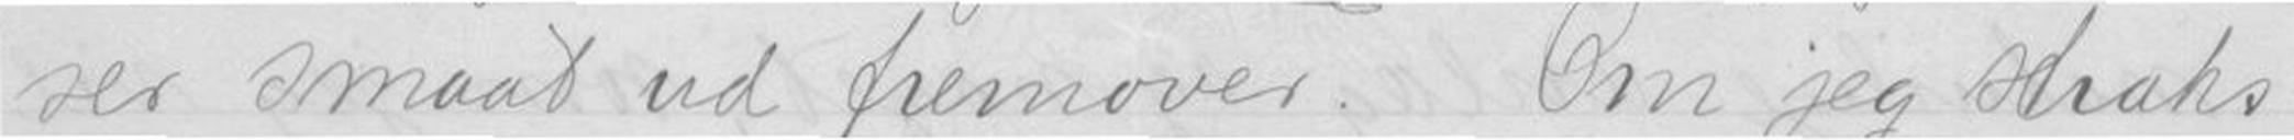ser smaat ud fremover. Om jeg straks
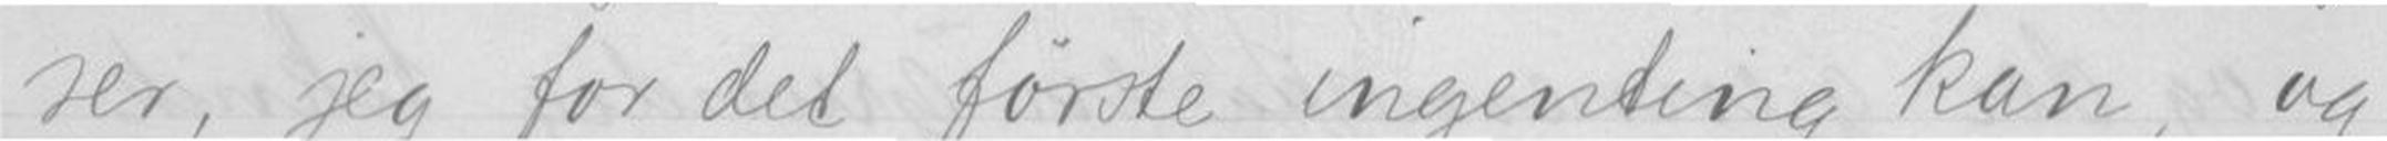ser, jeg for det første ingenting kan, og
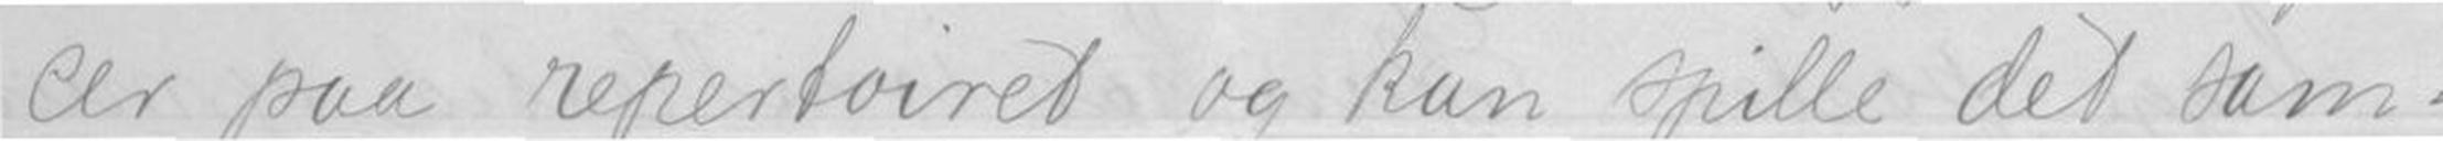cer paa repertoiret og kun spille det sam-
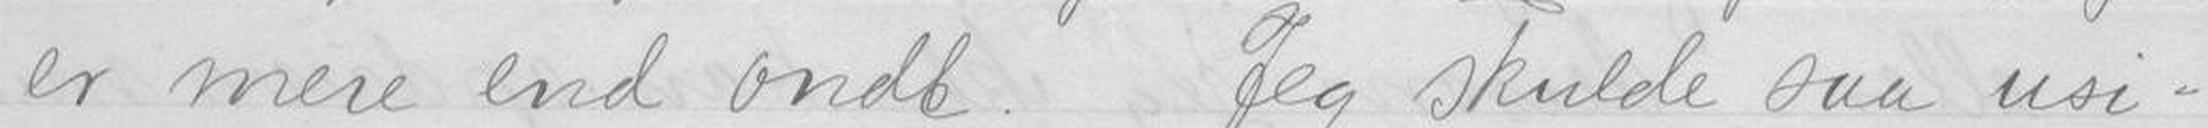er mere end ondt. Jeg skulde saa usi-
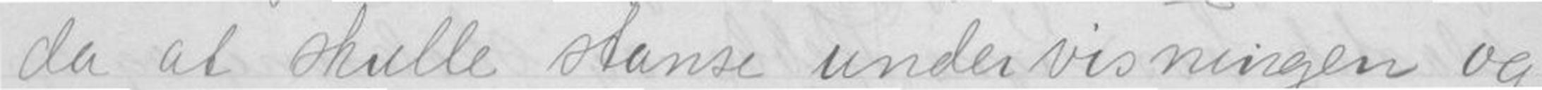da at skulle stanse undervisningen og
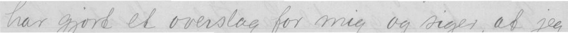har gjort et overslag for mig og siger, at jeg
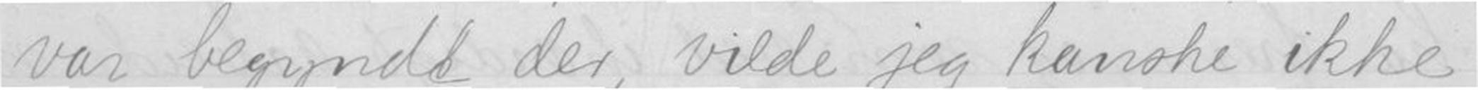var begyndt der, vilde jeg kanske ikke
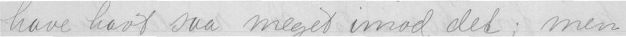have havt saa meget imod det; men
